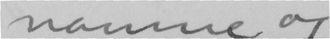namne, og
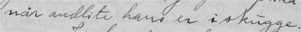når andlite hans er i skugge.
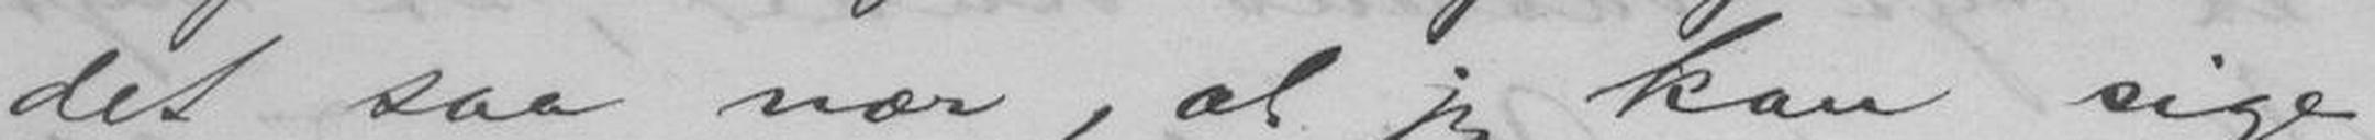det saa nær, at jeg kan sige
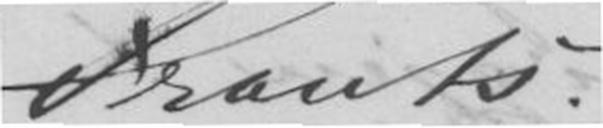Frants.
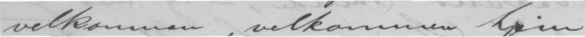velkommen, velkommen hjem
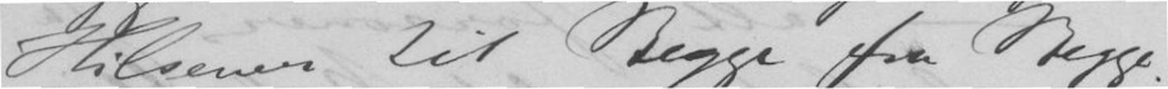Hilsener til Begge fra Begge!
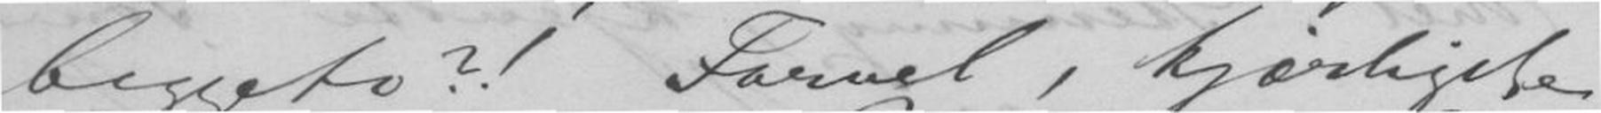beggeto?! Farvel, kjærligste
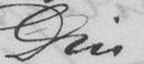Din
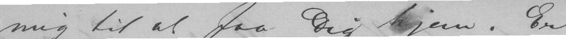mig til at faa Dig hjem. Er
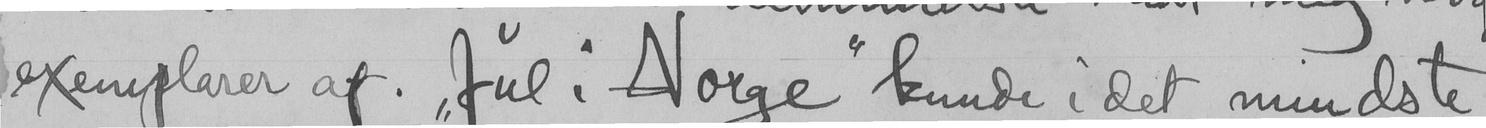exemplarer af. "Jul i Norge" kunde i det mindste
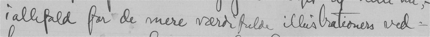iallefald for de mere værdifulde illustrationers ved-
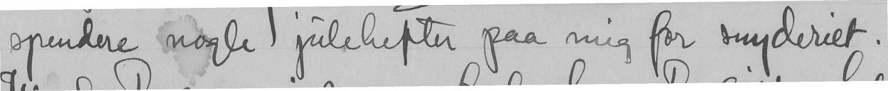spendere nogle julehefter paa mig for snyderiet.
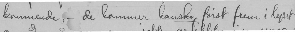kommende; - de kommer kanske først frem i lyset
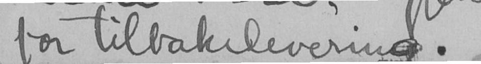for tilbakelevering.
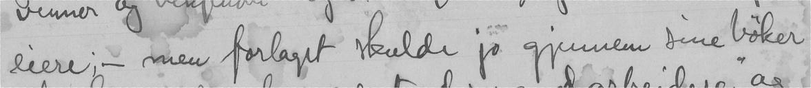eiere, - men forlaget skulde jo gjennem sine bøker
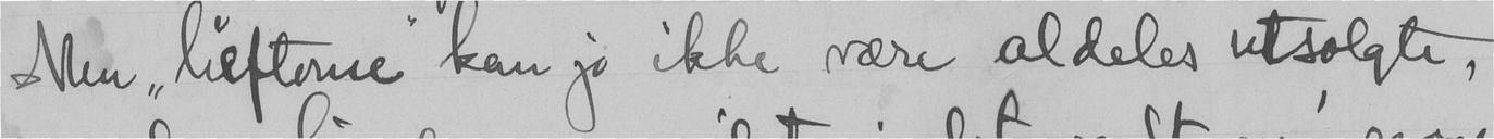Men "hefterne" kan jo ikke være aldeles utsolgte,
